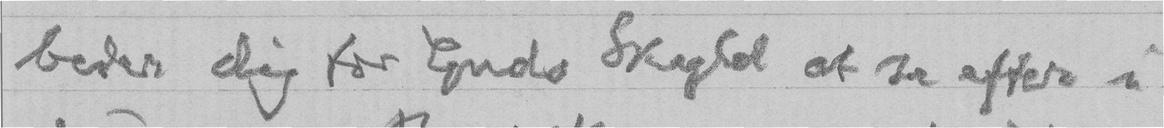beder dig for Guds Skyld at se efter i
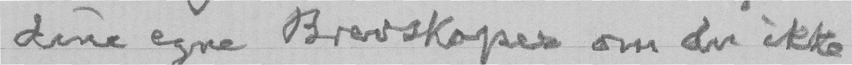dine egne Brevskaper om du ikke
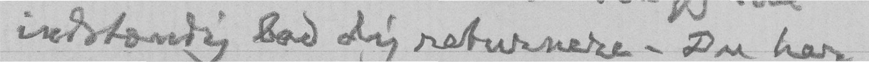indstændig bad dig returnere. Du har
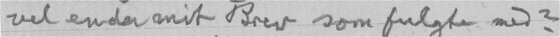vel enda mit Brev som fulgte med?
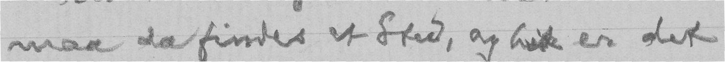maa da findes et Sted, og hit er det
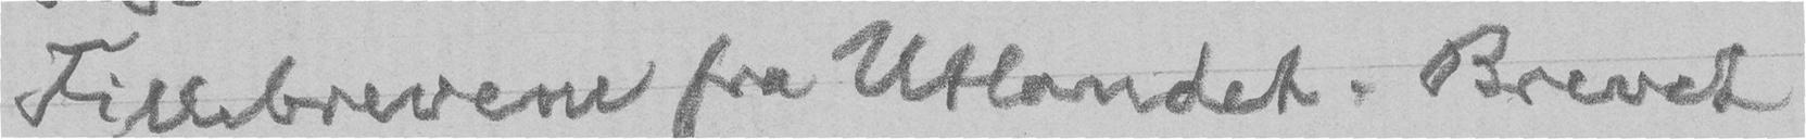Fillebrevene fra Utlandet. Brevet
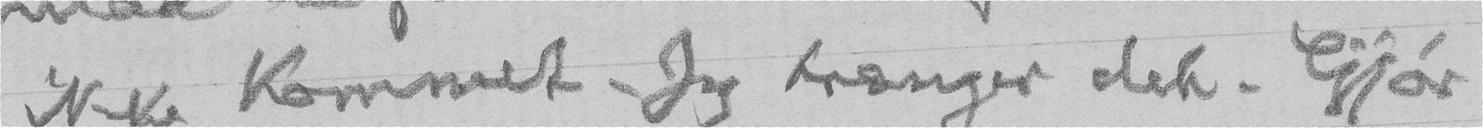ikke kommet. Jeg trænger det. Gjør
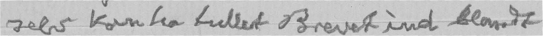selv kan ha tullet Brevet ind blandt
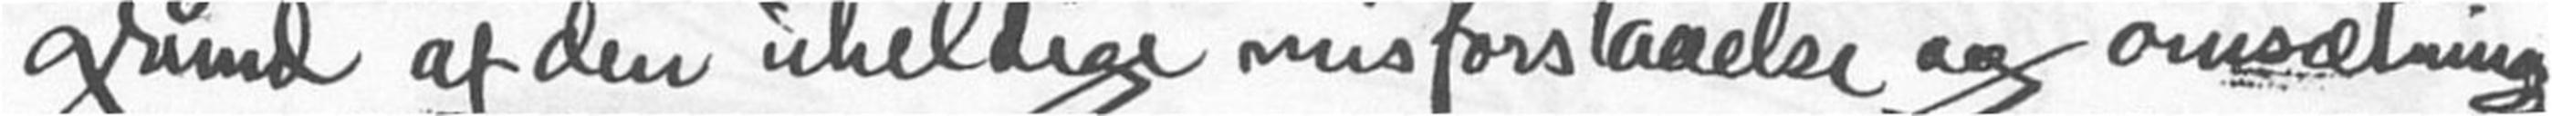grund af den uheldige misforstaaelse og omsætning
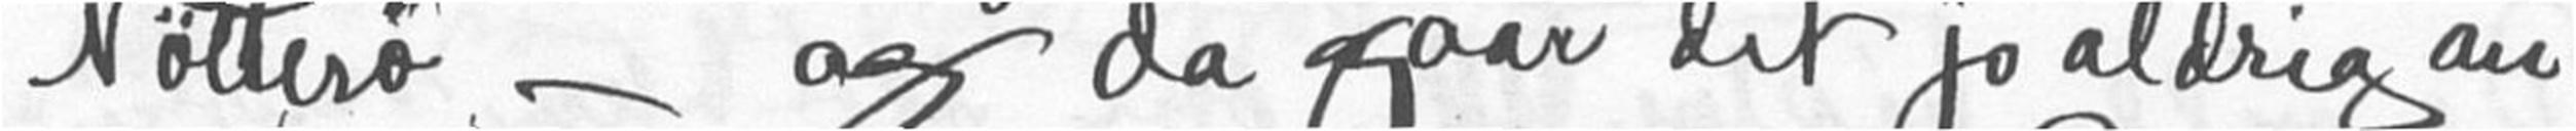Nøtterø - og da gaar det jo aldrig an
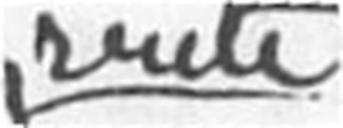rente
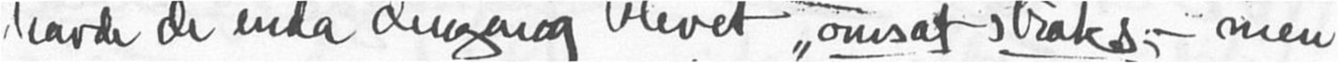havde de enda dengang blevet "omsat" straks, - men
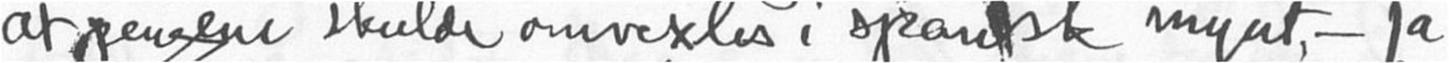at pengene skulde omvexles i spansk mynt, - ja
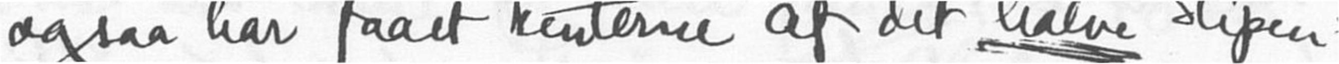ogsaa har faaet renterne af det halve stipen-
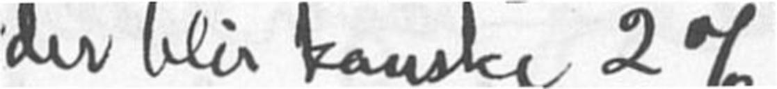der blir kanske 2%
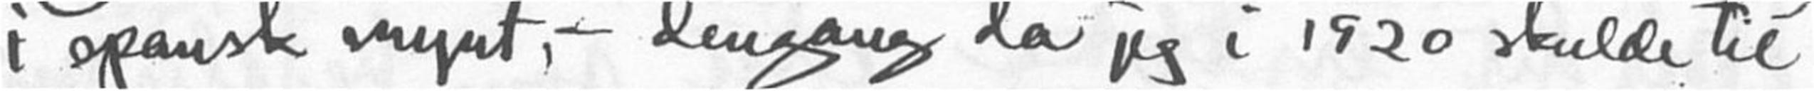i spansk mynt, - dengang da jeg i 1920 skulde til
